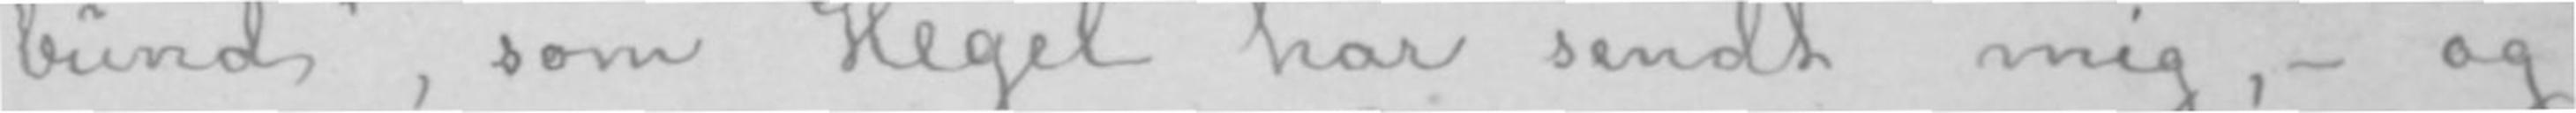bund», som Hegel har sendt mig,- og
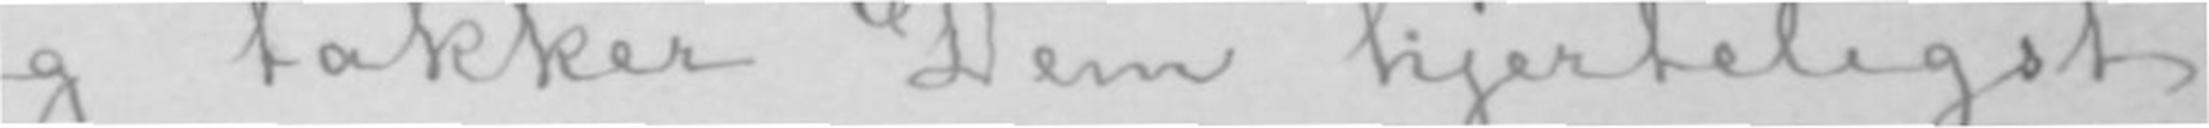Jeg takker Dem hjerteligst
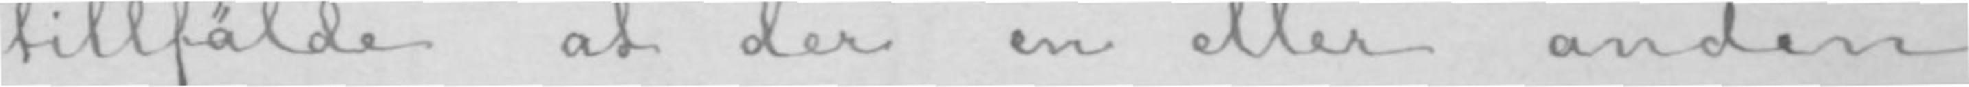tillfälde at der en eller anden
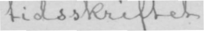tidsskriftet.
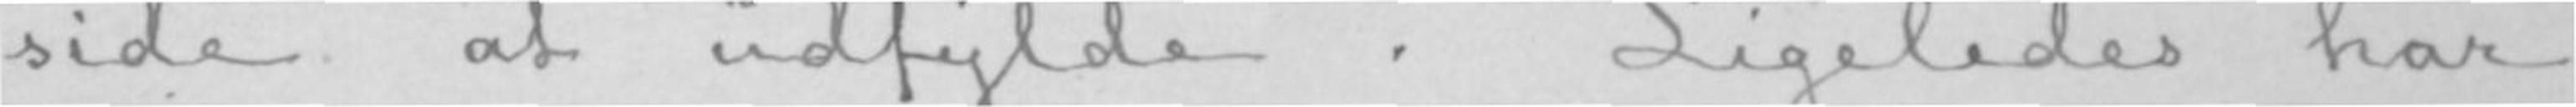side at udfylde. Ligeledes har
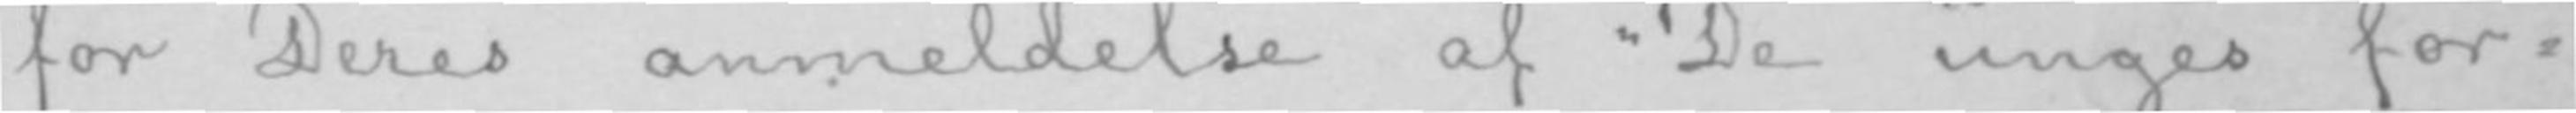for Deres anmeldelse af «De unges for-
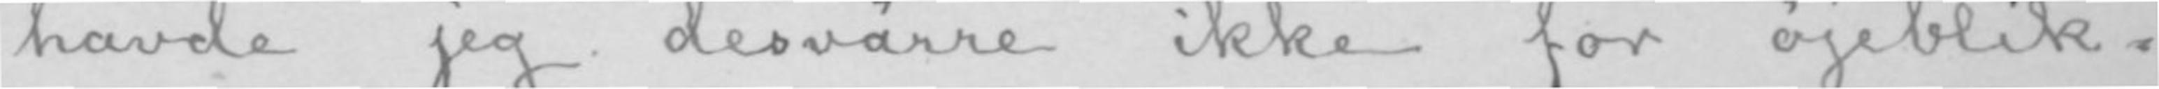havde jeg desvärre ikke for öjeblik-
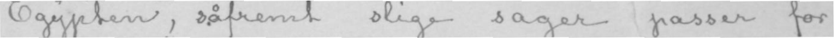Egypten, såfremt slige sager passer for
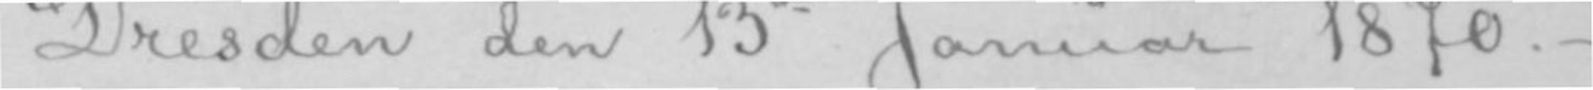Dresden den 13de Januar 1870.-
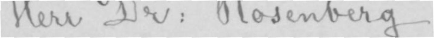Herr Dr: Rosenberg!
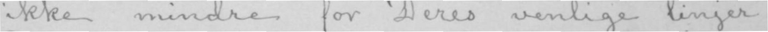ikke mindre for Deres venlige linjer.
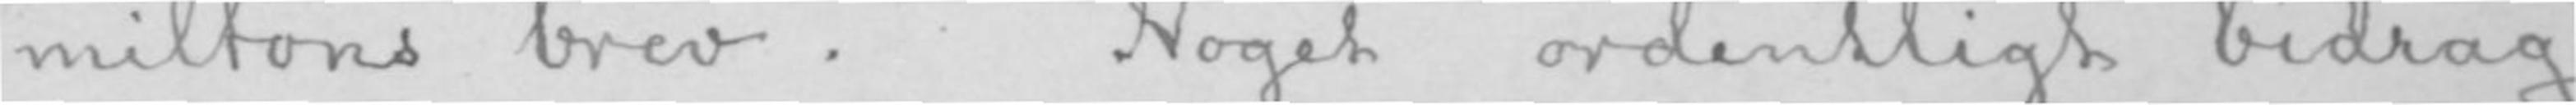miltons brev. Noget ordentligt bidrag
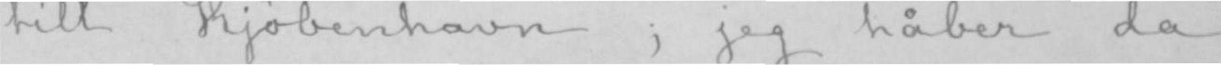till Kjöbenhavn; jeg håber da
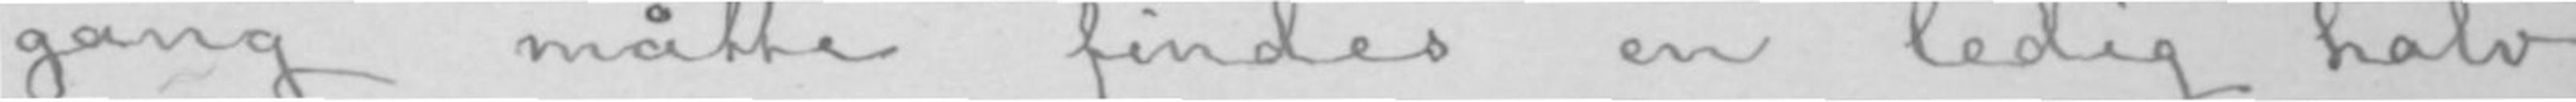gang måtte findes en ledig halv
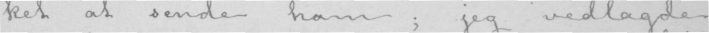ket at sende ham; jeg vedlagde
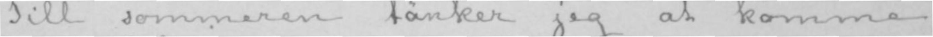Till sommeren tänker jeg at komme
